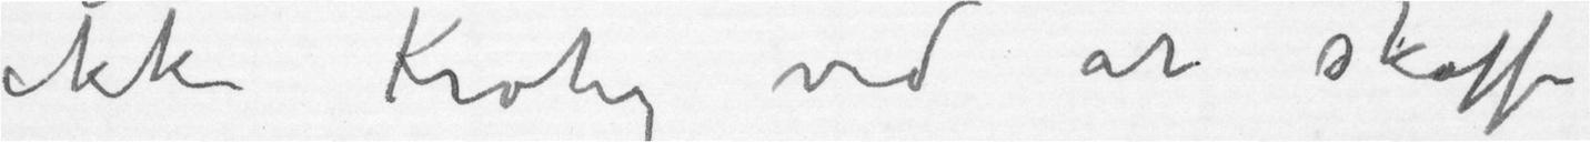ikke Krohg ved at skaffe
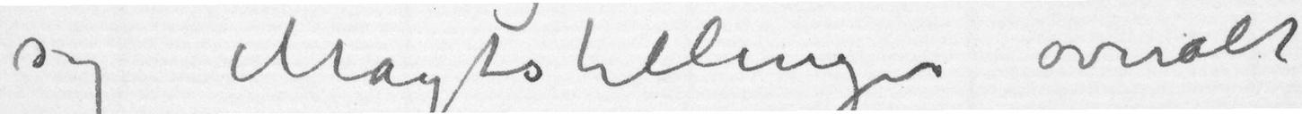sig Magtstillinger overalt
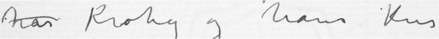har Krohg og hans Kres
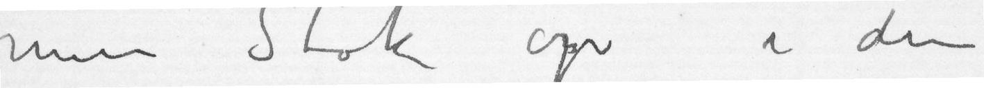min Stok op i den
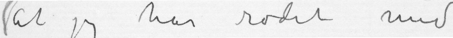(at jeg har rodet med
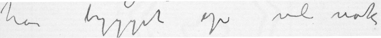har bygget op vil nok
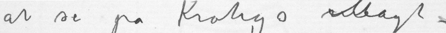at se på Kroghs Magt -
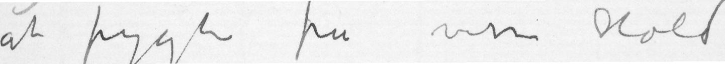at frygte fra visse Hold
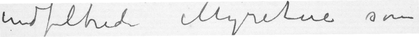indfulhede Myretue som
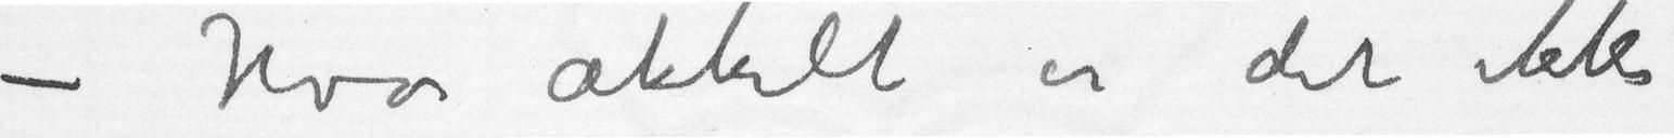– Hvor ækkelt er det ikke
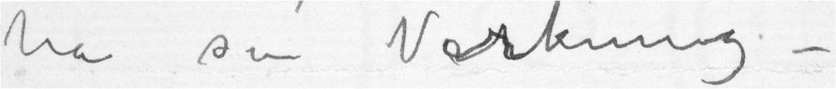ha sin Virkning –
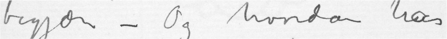begjær – Og hvordan har
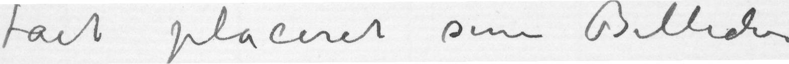fået placeret sine Billeder
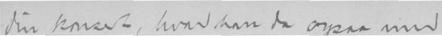din konsert, hvad han da ogsaa med
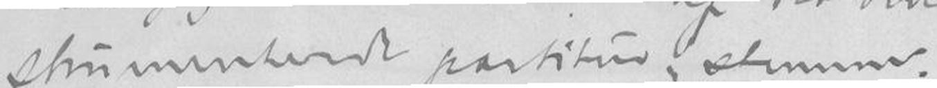strumenterede partitur og stemmer.
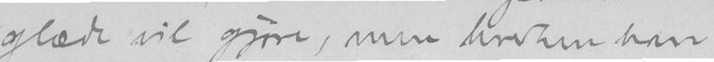glæde vil gjøre, men hvordan han
